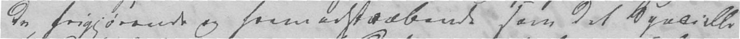de frigjørende og fremadstræbende som det Specielle
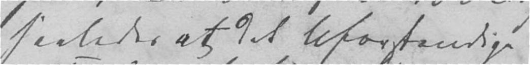saaledes at det Uforstandige
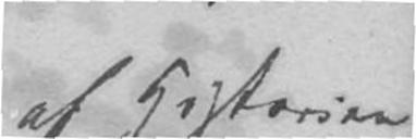af Historien
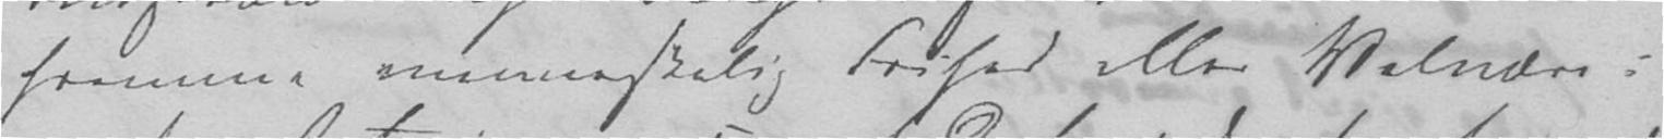fremme menneskelig Frihed eller Velvære i
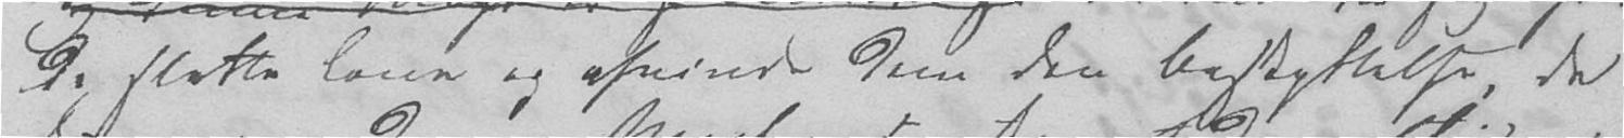de slette Love og afvinde dem den Beskyttelse de
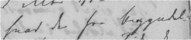hvad der fra Begyndel-
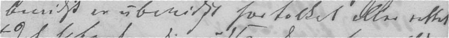bevidst ev ubevidst fortolket eller rettet
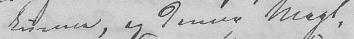kunne, og denne Magt,
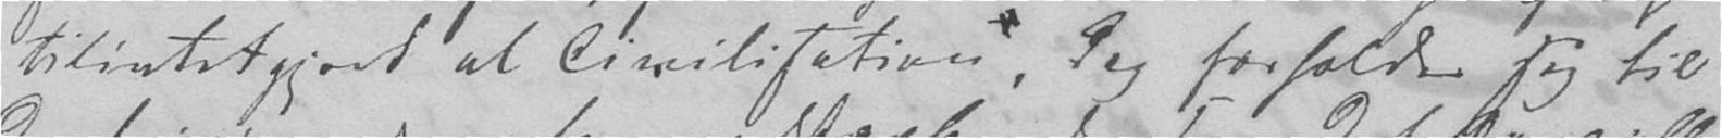tilintetgjort al Civilisation, dog forholder sig til
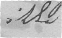ikke
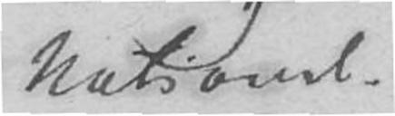National-
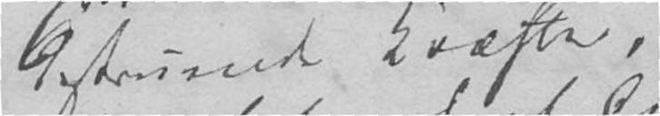destruende Kræfter,
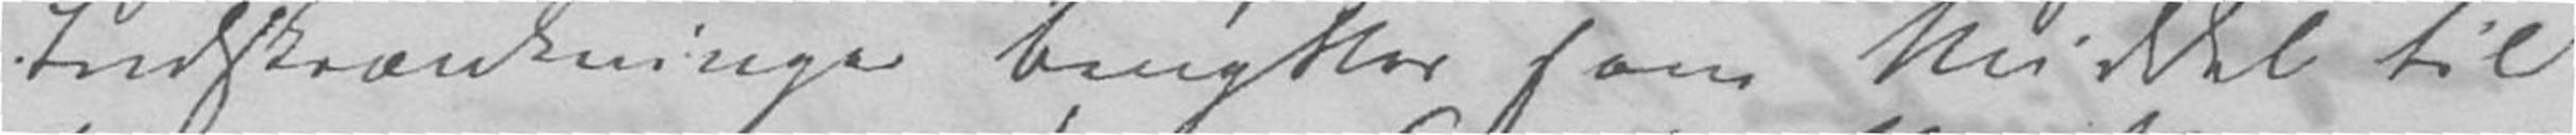Indskrænkninger benyttes som Middel til
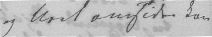og Uret omsider kan
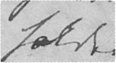holde
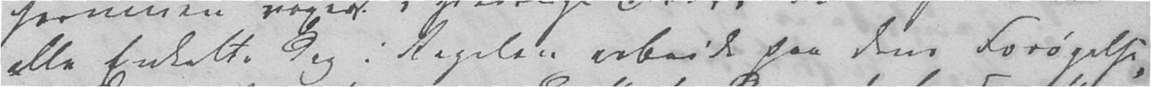alle Enkelte dog i Regelen arbeide paa dens Forøgelse.
